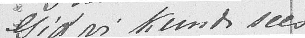Gid vi kunde sees
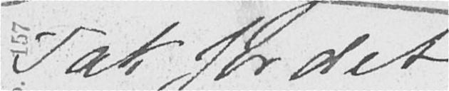Tak for det
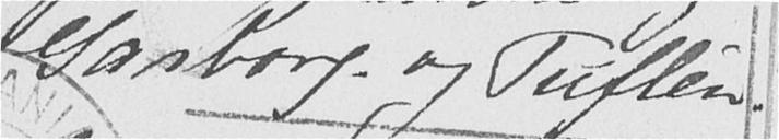Garborg og Tuften.
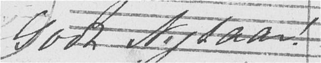Godt Nytaar!
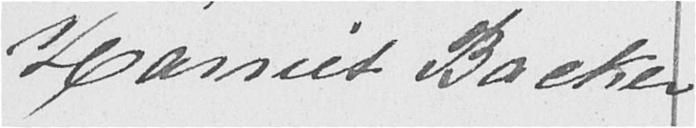Harriet Backer
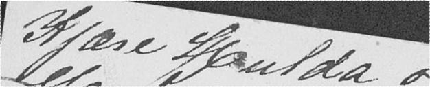Kjære Hulda og
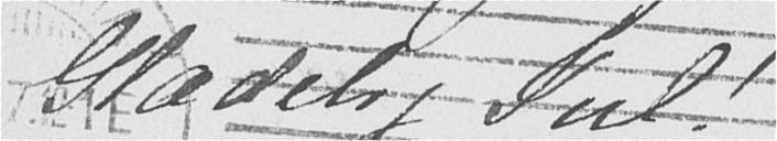Glædelig Jul!
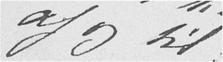af og til.
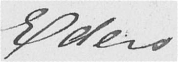Eders
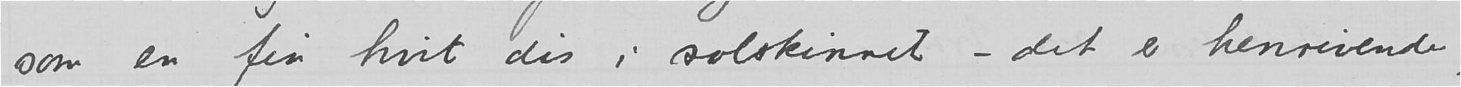som en fin hvit dis i solskinnet – det er henrivende
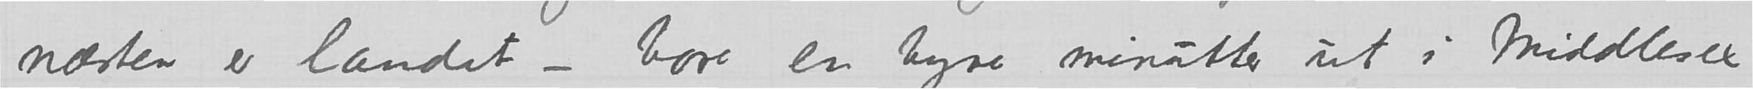næsten er landet – bare en tyve minutter ut i Middlesex
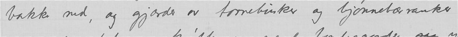bakke ned, og gjærder av tornebusker og bjørnebærranker
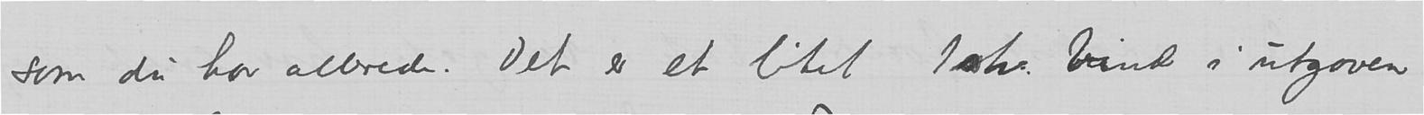som du har allerede. Det er et litet 1eks. bind i utgaven
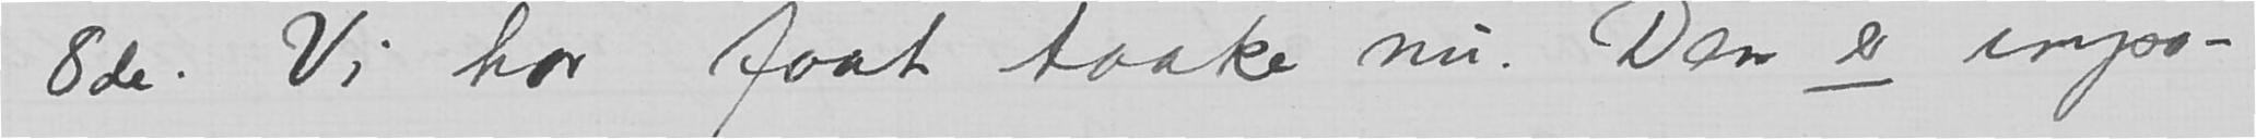8de. Vi har faat taake nu. Den er impo-
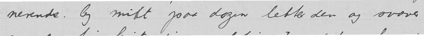nerende. Og mitt paa dagen letter den og svæver
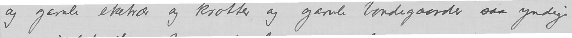og gamle eketrær og krøtter og gamle bondegaarder saa yndige
